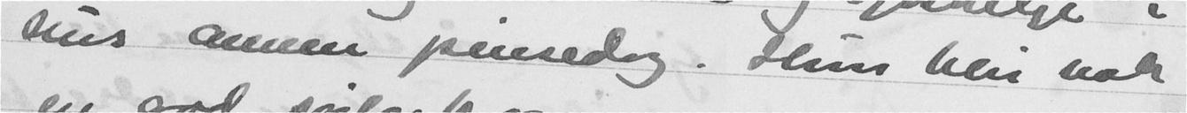hus annen pinsedag. Hun blir nok
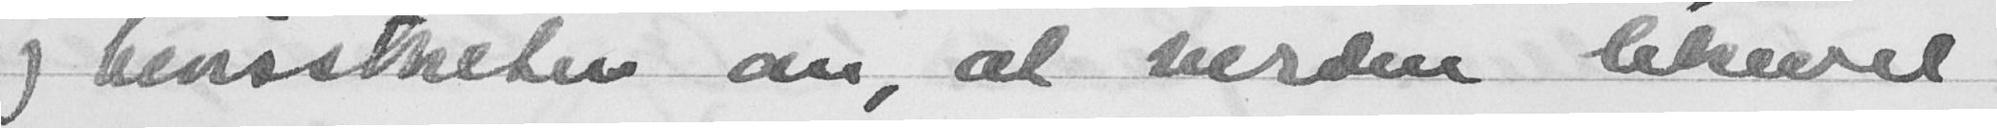og bevisstheten om, at verden likevel
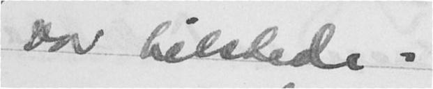var tilstede.
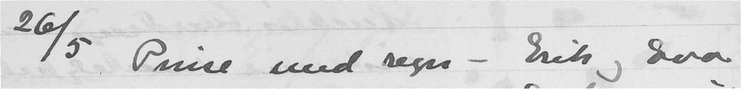26/5 Pinse med regn - Erik og Eva
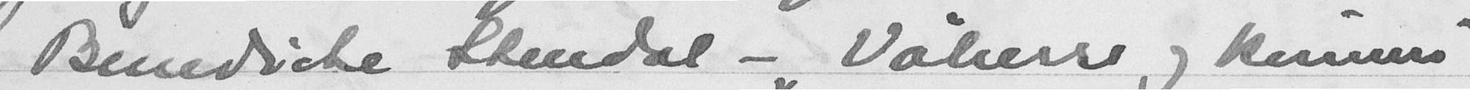Benedicte Stendal - "Vårherre og kvinne"
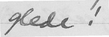glede!
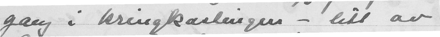gang i kringkastingen - litt av
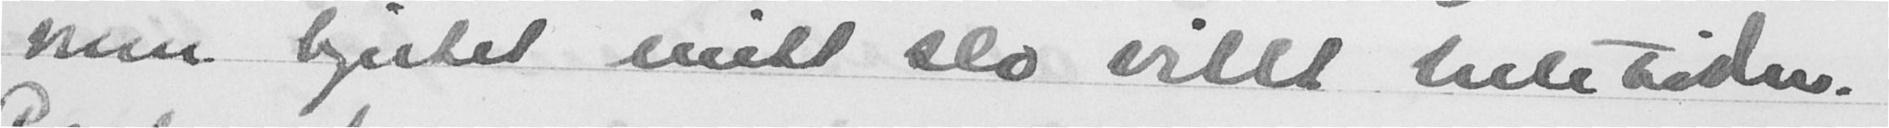men hjertet mitt slo villt hele tiden.
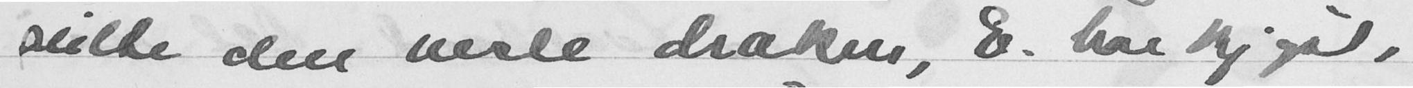seilte den vesle draken, E. har kjøpt,
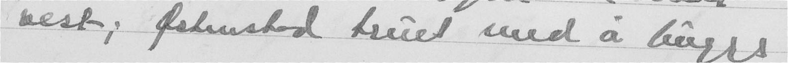vest; fstenstad truet med å bygge
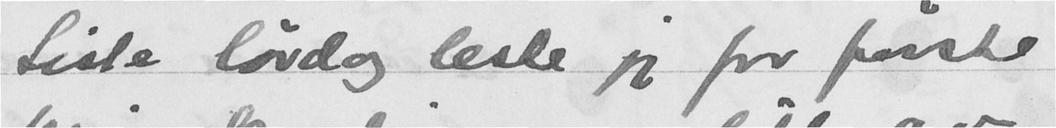Siste lørdag leste jeg for første
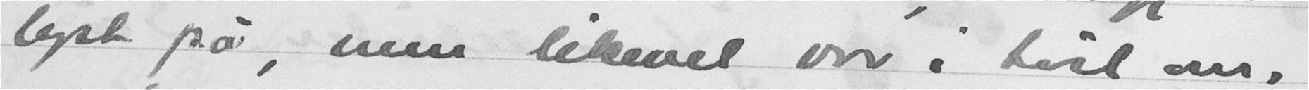lyst på, men likevet var i tvil om,
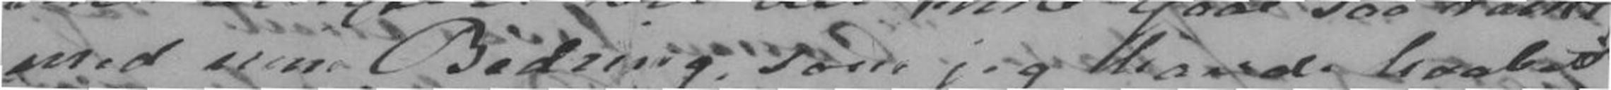med min Bedring, som jeg havde haabet
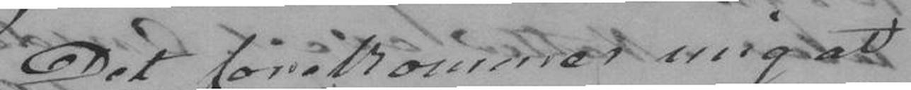Det forekommer mig at
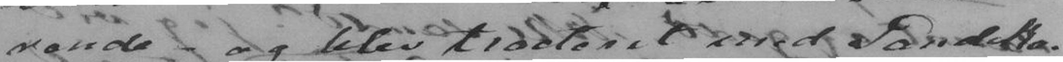rende - og blev trakteret med Pandeka-
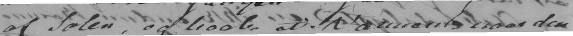af Solen, og haabe at Varmen naar den
